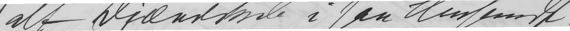alt Djævelskab i saa Henseende. -
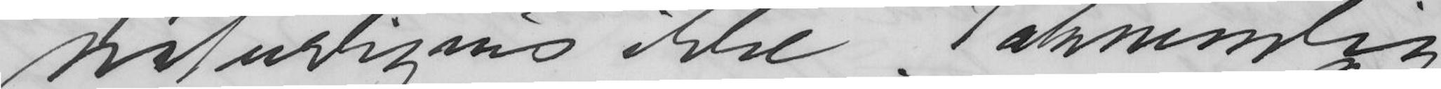naturligvis ikke. Taknemlig
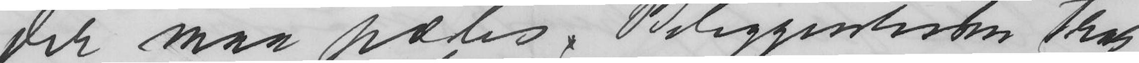der maa pæles. Beliggenheden tror
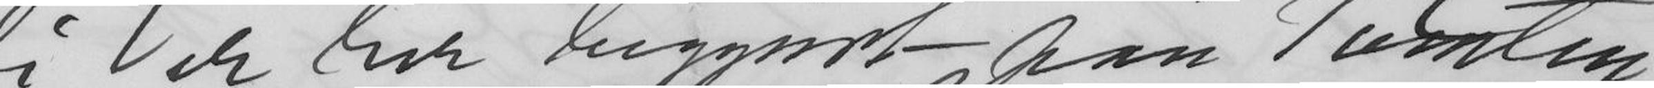Vi er her begyndt paa Tomten
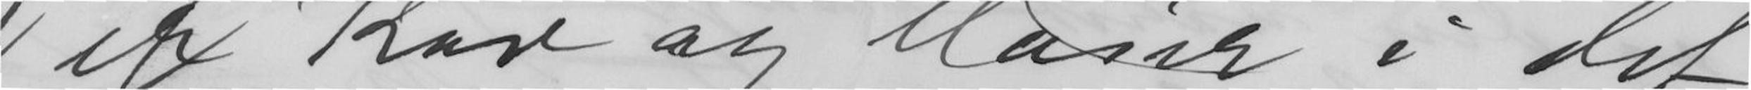er Kav og Møier i det
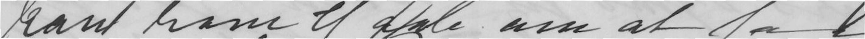kan have Haab om at faa
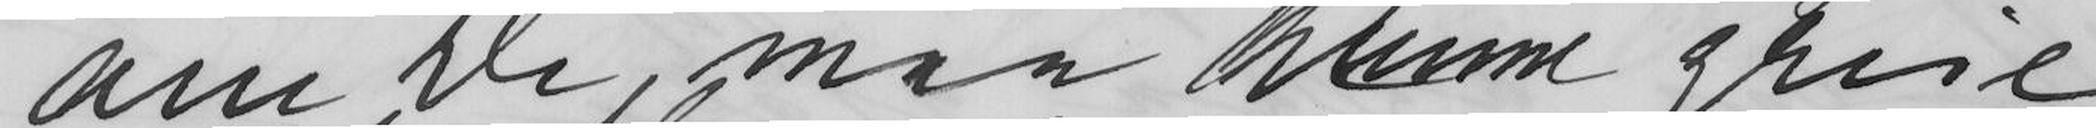om De maa kunne greie
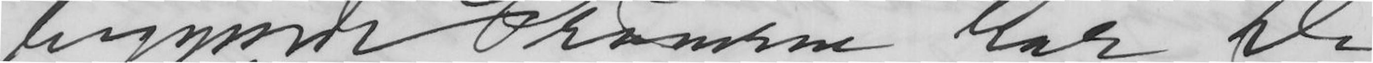begynde Prøvene har De
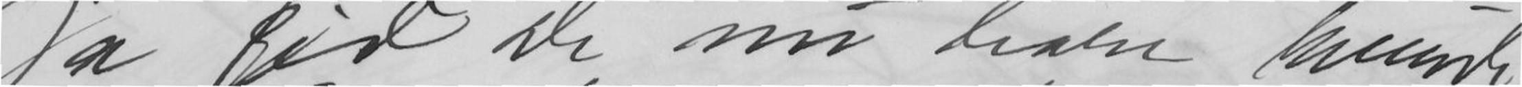Ja Gid De nu bare kunde
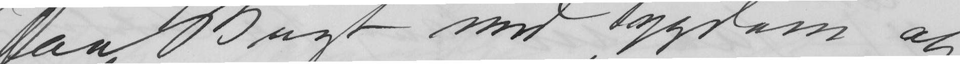faa Bugt med Sygdom og
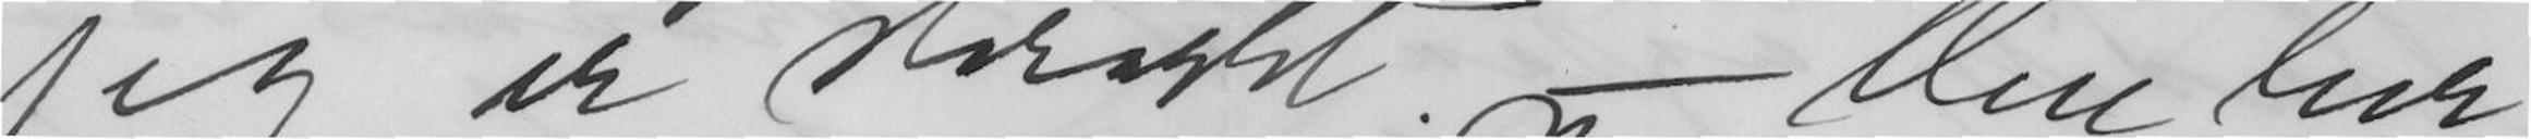jeg er storartet. - Men her
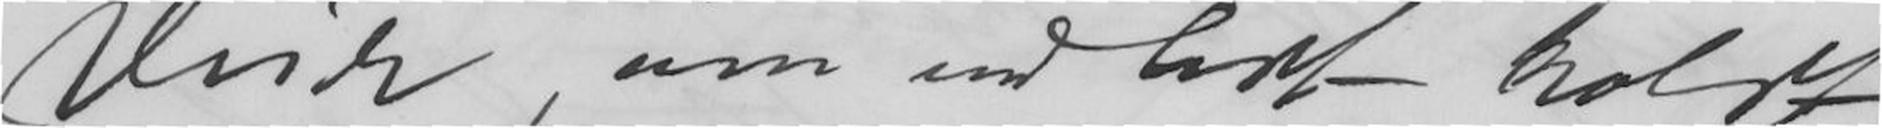Veir, om end lidt koldt. -
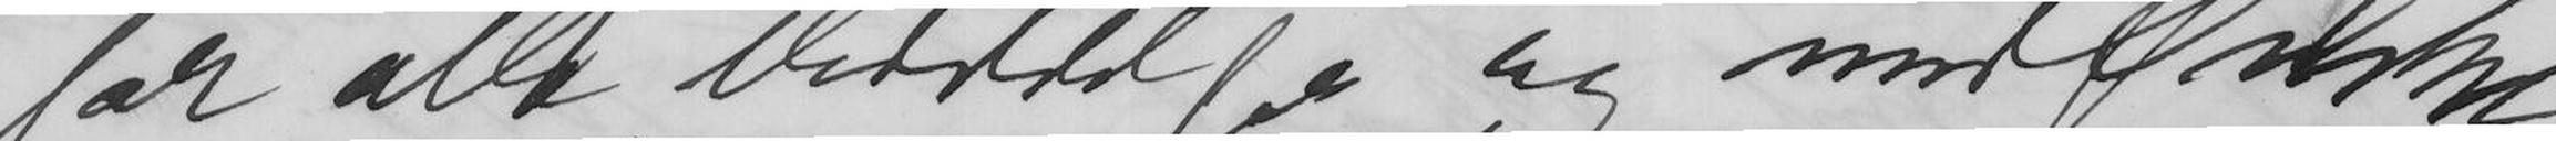for all Meddelelse og med Ønske
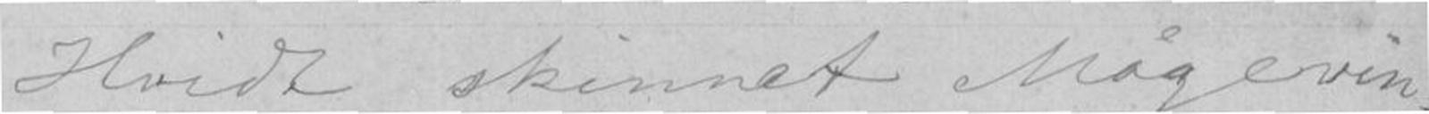Hvidt skinnet Mågevin-
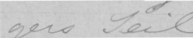gers Seil
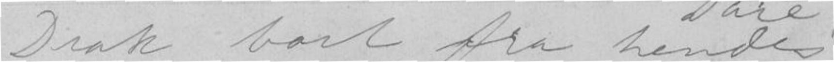Drak bort fra hendes
